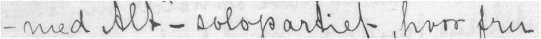- med Alt - solopartiet, hvor fru
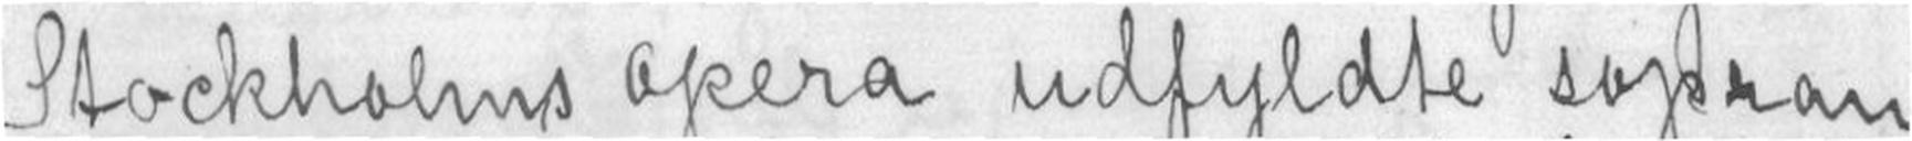Stockholms opera udfyldte sopran
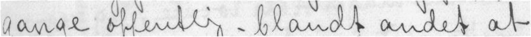gange offentlig - blandt andet at
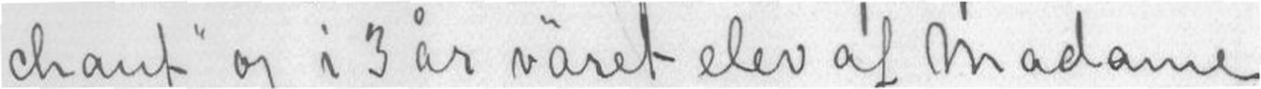chant og i 3 år väret elev af Madame
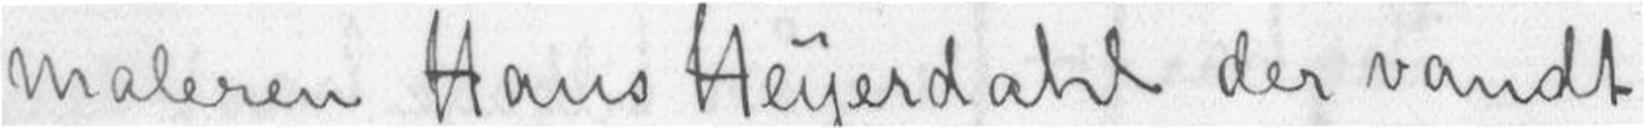maleren Hans Heyerdahl der vandt
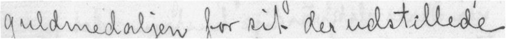guldmedaljen for sit der udstillede
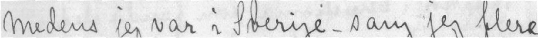Medens jeg var i Sverige sang jeg flere
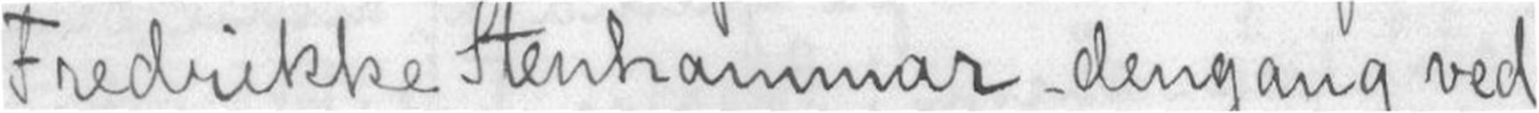Fredrikke Stenhammar - dengang ved
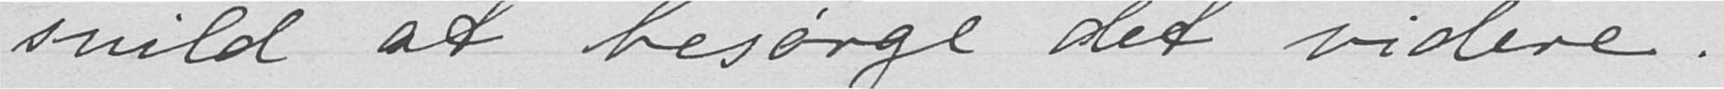snild at besørge det videre.
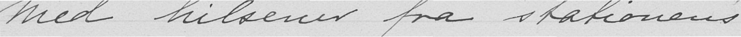Med hilsener fra stationens
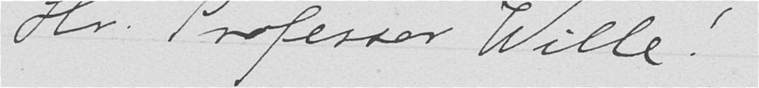Hr. Professor Wille!
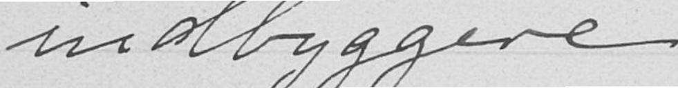indbyggere
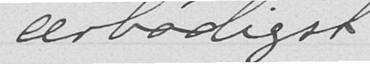ærbødigst
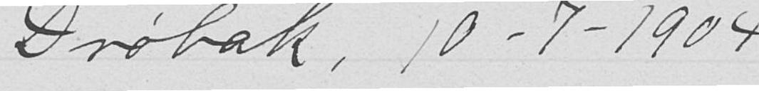Drøbak, 10 - 7 - 1904
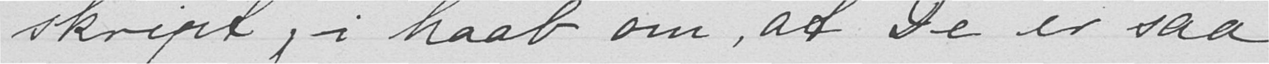skript, i haab om, at De er saa
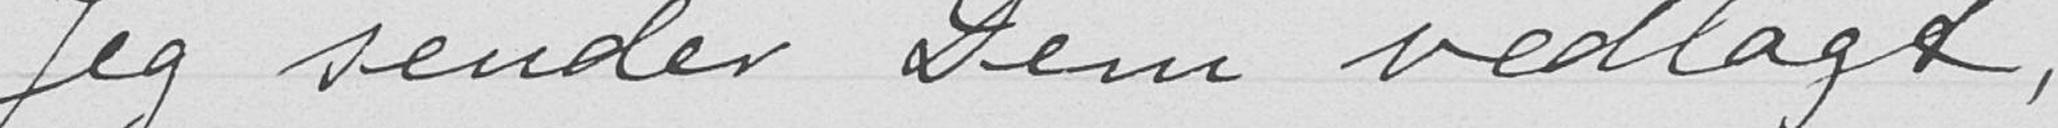Jeg sender Dem vedlagt,
